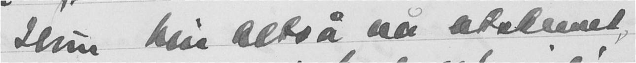Hun blir altså nå atelemet,
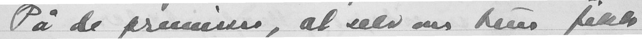På de premisser, at selv om hun fikk
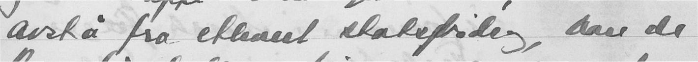avstå fra ethvert statsfridrag, bare de
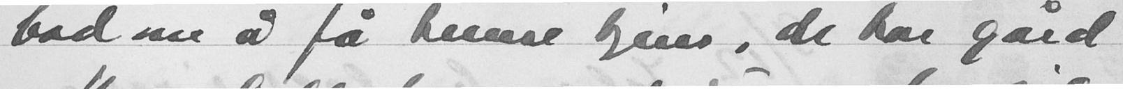bad om a få henne hjem, de har gård
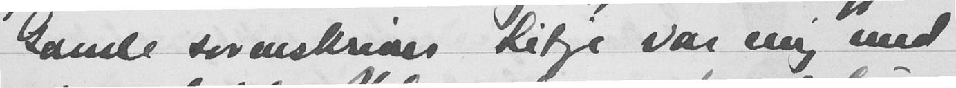Gamle sorenskriver Kitje var enig med
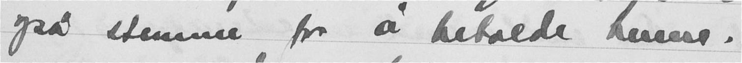også stemme før å beholde henne.
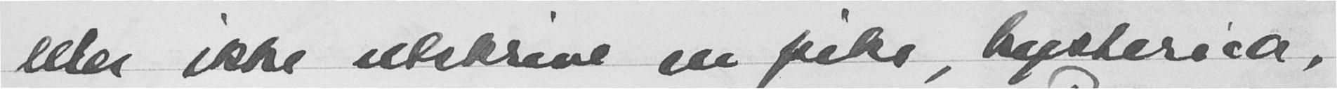eller ikke utskrive en pike, hysterica,
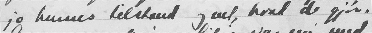jo hennes tilstand og vet, hvad de gjør.
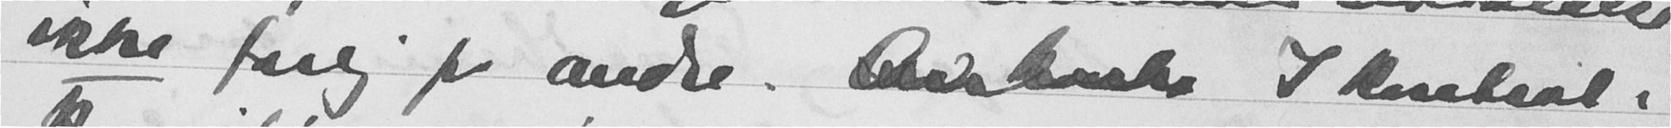ikke farlig for andre. Overlege J Rinkval.
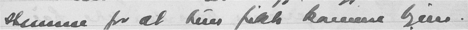stemme for at hun fikk komme hjem.
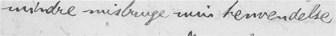mindre misbruge min henvendelse.
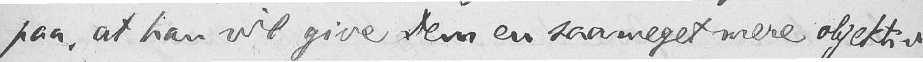paa, at han vil give Dem en saameget mere objektiv
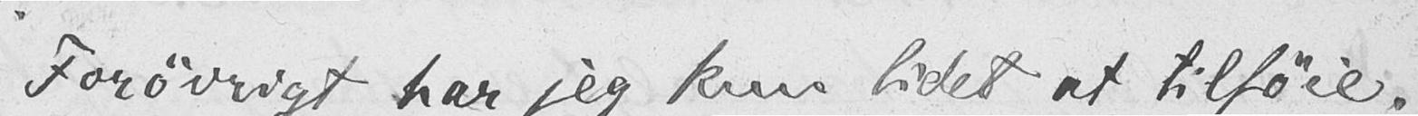Forøvrigt har jeg kun lidet at tilføie.
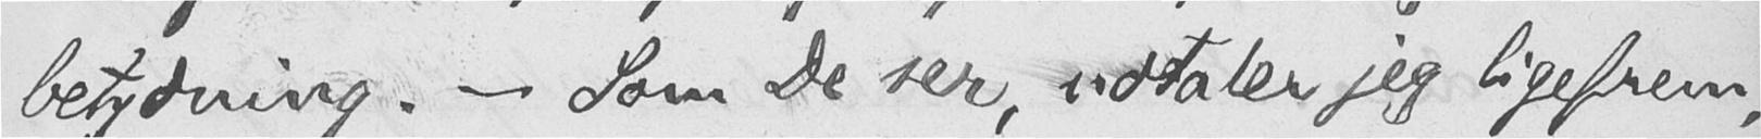betydning. - Som De ser, udtaler jeg ligefrem,
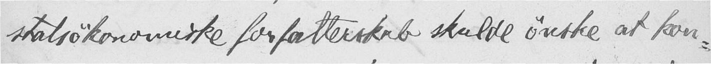statsøkonomiske forfatterskab skulde ønske at kon-
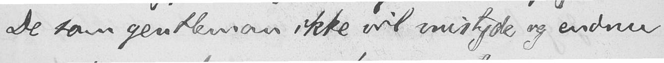De som gentleman ikke vil mistyde og endnu
